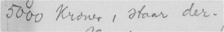5000 Kroner, staar der.
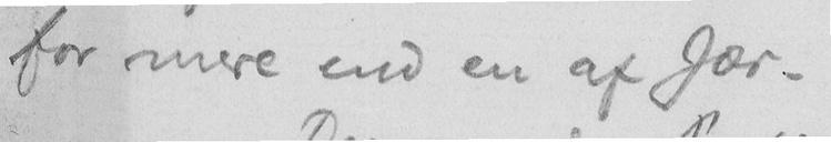for mere end en af Jær.
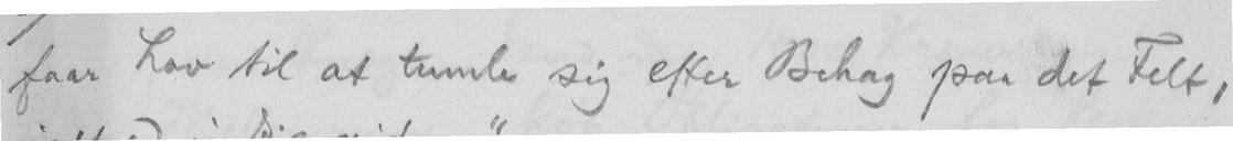faar Lov til at tumle sig efter Behag paa det Felt,
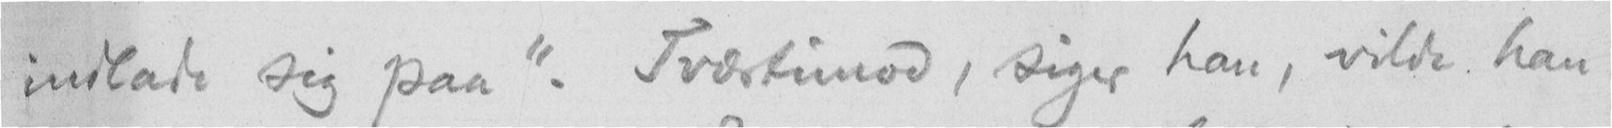indlade sig paa". Tværtimod, siger han, vilde han
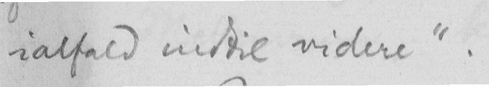ialfald indtil videre".
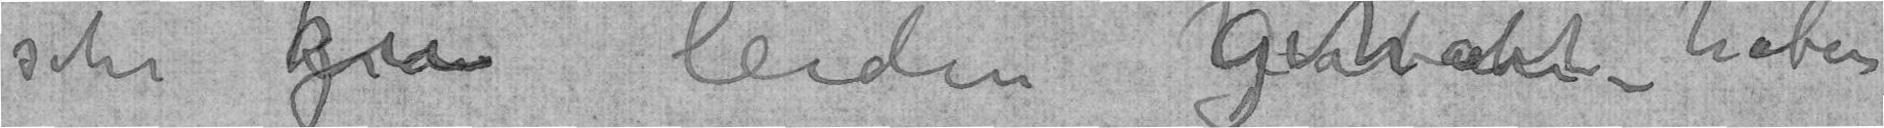sehrleiden gehabt haben
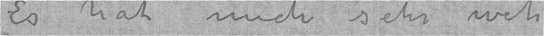Es hat mich sehr weh
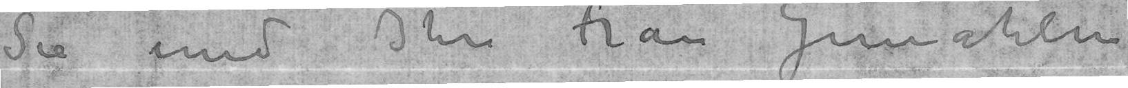Sie und Ihre Frau Gemahlin
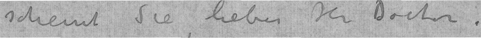scheint Sie lieben Hr Doctor.
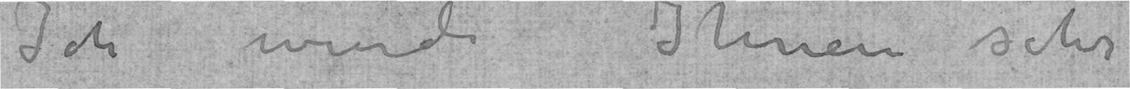Ich wurde Ihnen sehr
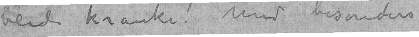beide kranke! Und besonders
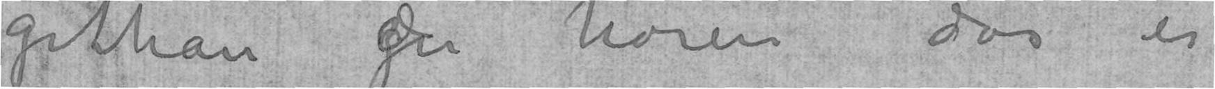gethan zu horen das es
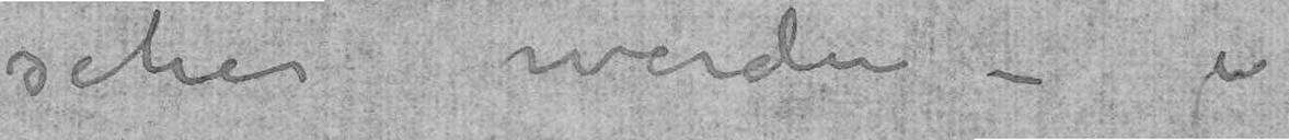sehen werden –
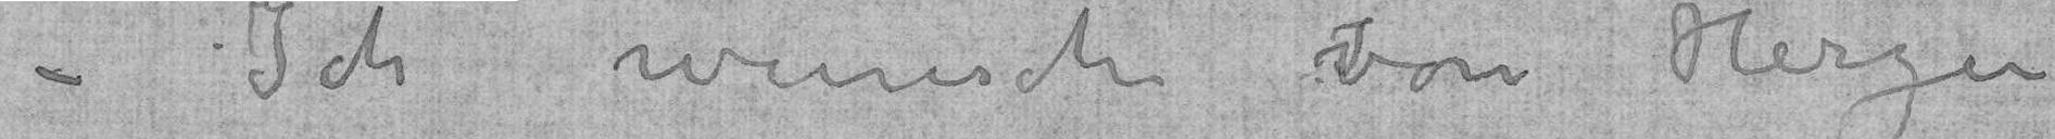– Ich wunsche von Herzen
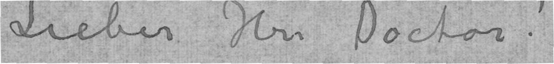Lieber Hrr Doctor!
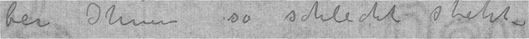bei Ihnen so schlecht steht –
